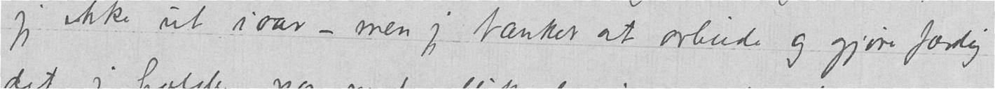jeg ikke ut iaar - men jeg tænker at arbeide og gjøre færdig
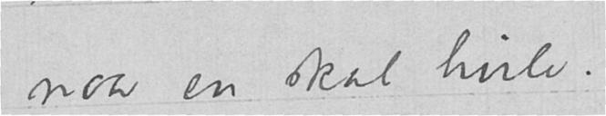naar en skal hvile.
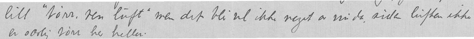litt «tørr, ren luft» men det blir vel ikke noget av nu da, siden luften ikke
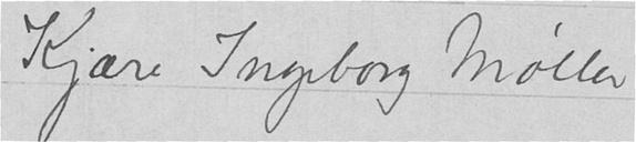Kjære Ingeborg Møller,
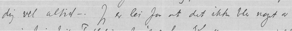dig vel altid –. Jeg er lei for at det ikke blev noget av
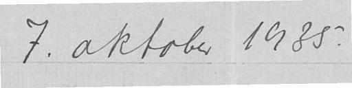7. oktober 1935.
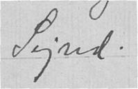Sigrid.
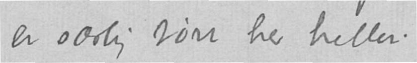er særlig tørr her heller.
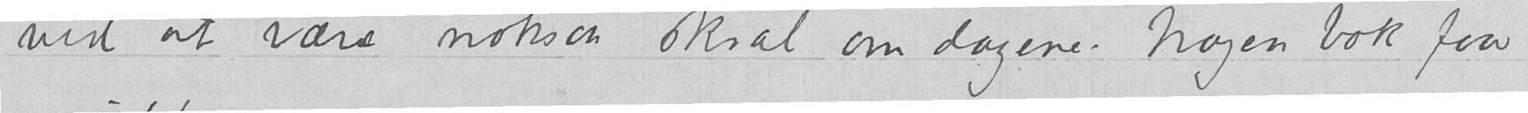ved at være noksaa skral om dagene. Nogen bok faar
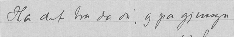Ha det bra da du, og paa gjensyn
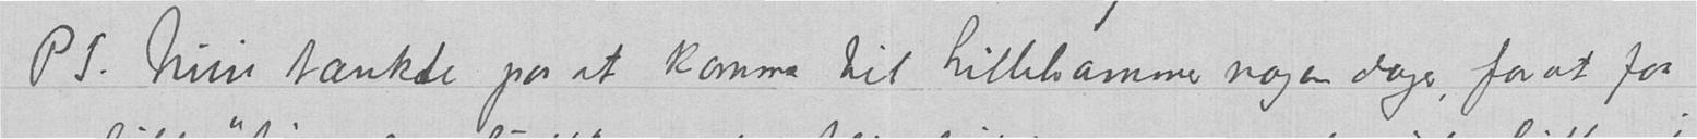PS. Nini tænkte paa at komme til Lillehammer nogen dager, for at faa
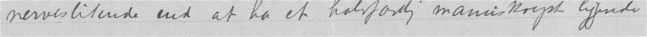nerveslitende end at ha et halvfærdig manuskript liggende
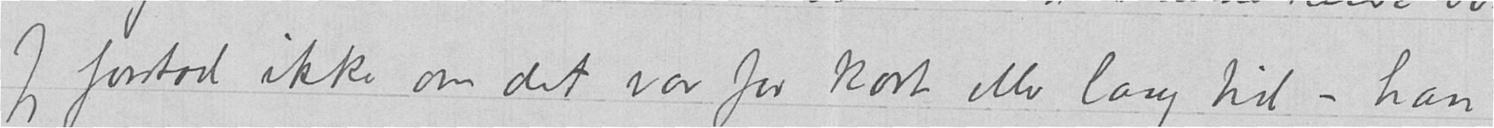Jeg forstod ikke om det var for kort eller lang tid – han
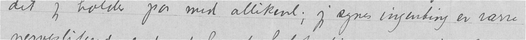det jeg holder paa med allikevel; jeg synes ingenting er værre
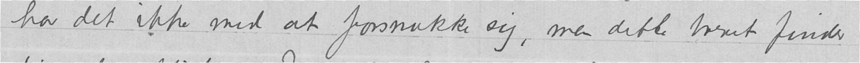har det ikke med at forsnakke sig, men dette brevet finder
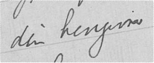din hengivne
